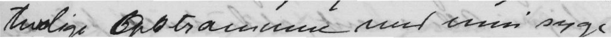turlige Opstramme med min syge
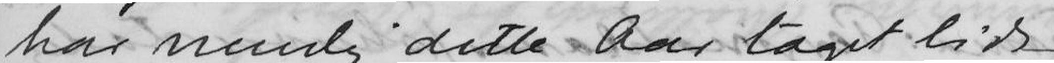har nemlig dette Aar taget lidt
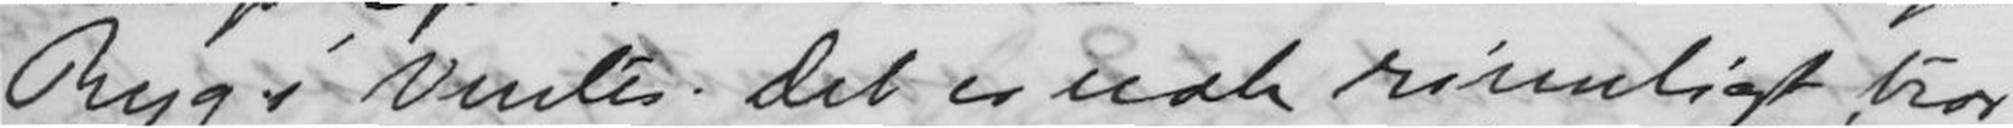Ryg i Vinter. Det er nok rimeligt tror
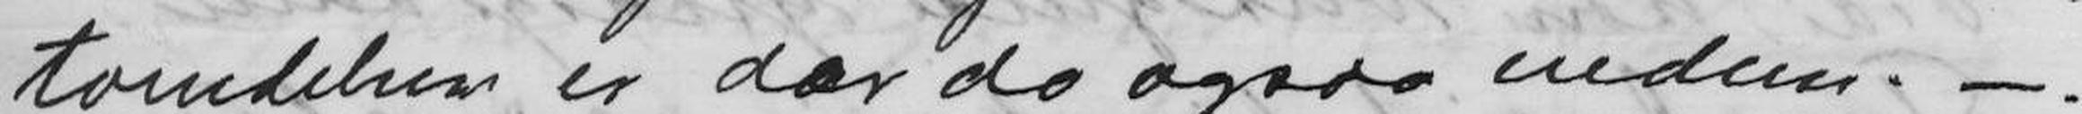taendelser er der da ogsaa endnu. -
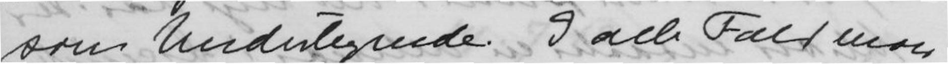som Undertegnede. I alle Fald maer-
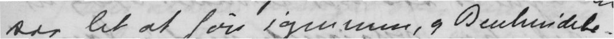saa let at føre igennem, og Benhinde-
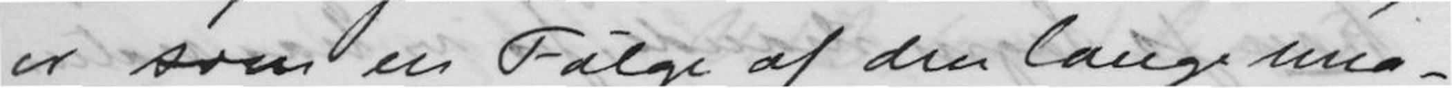er som en Følge af den lange una-
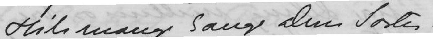Hils mange Gange Deres Søster.
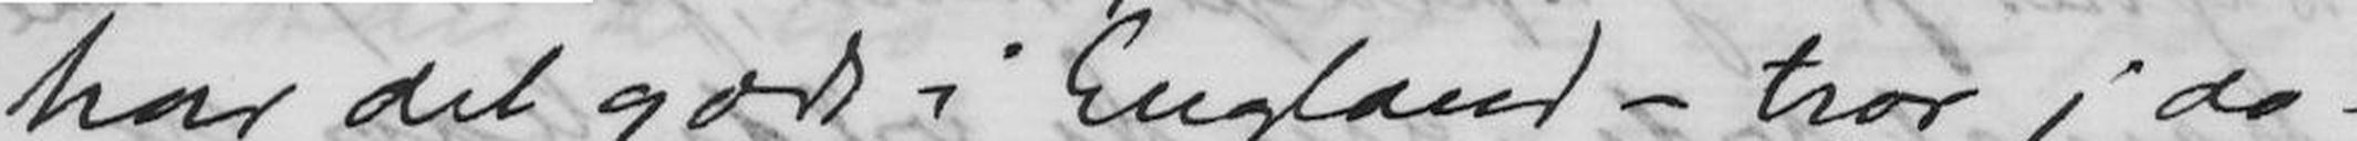har det godt i England - tror j da -
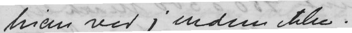hiem ved j endnu ikke.
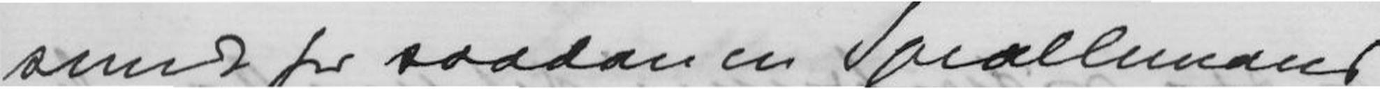sundt for saadan en Spraellemand
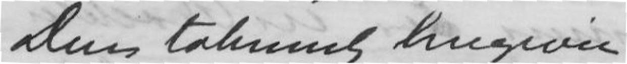Deres taknemlig hengivne
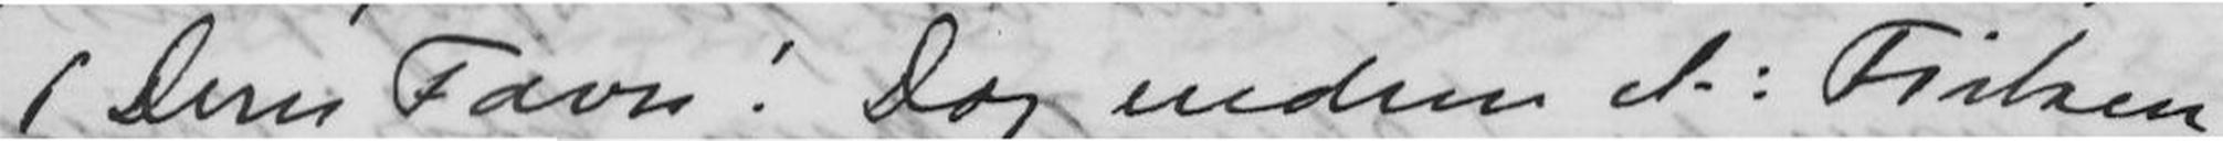i Deres Favn! Dog endnu et: Fisken
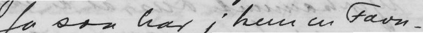Ja saa har j kun en favn-
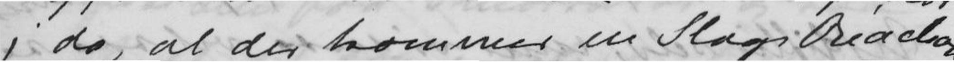j do, at der kommer en Slags Reaction
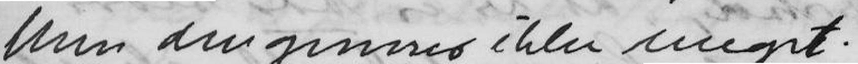Men den generer ikke meget.
